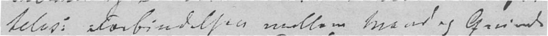teles. Forbindelsen mellom Mand og Qvinde
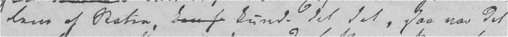lem af Staten. kan ha kunde det det, saa var det
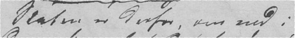Staten er derfor, om end i
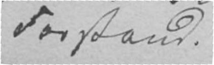Forstand.
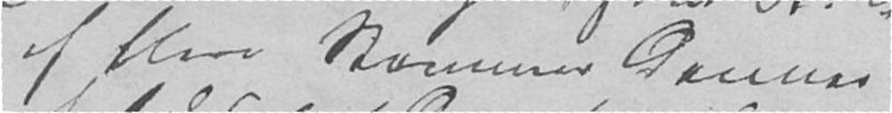af flere Stammer danner
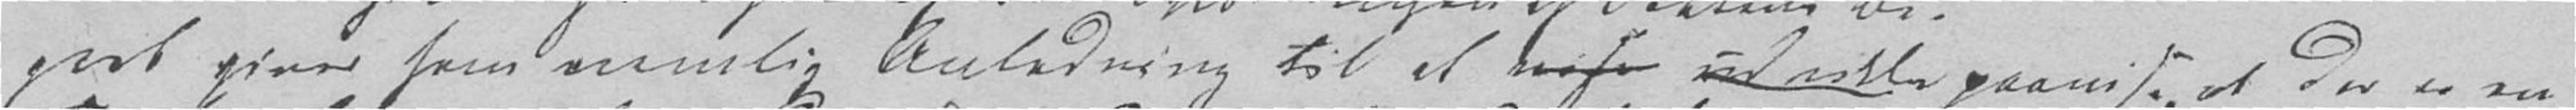greb giver ham nemlig Anledning til at vise udvikle paavise, at der er en
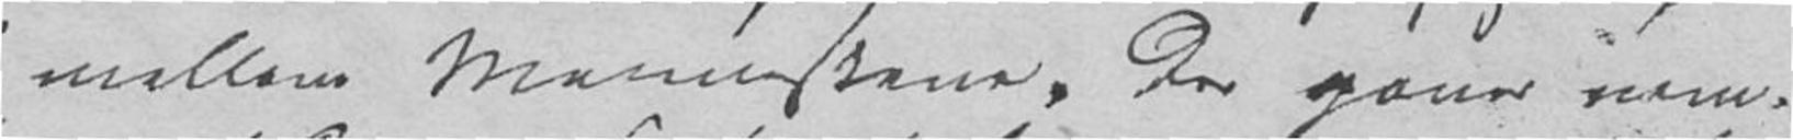mellem Menneskene. Der gaves nem-
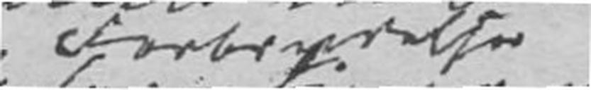Forbindelser
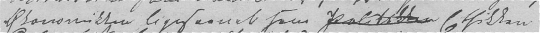Økonomikken ligesaavel som Politikken Ethikken
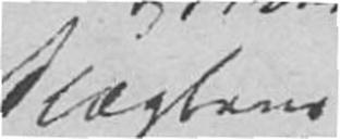Slægtens
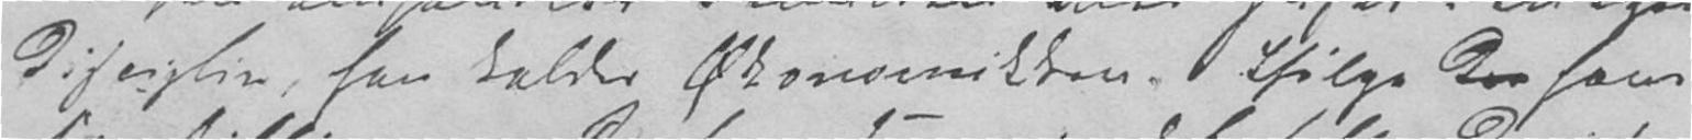Disicplin, han kalder Økonomikken. Ifølge den hans
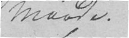Maade.
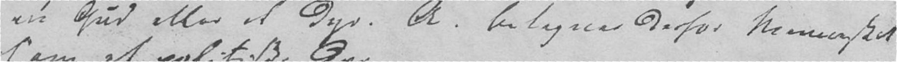en Gud eller et Dyr. A. betegner derfor Mennesket
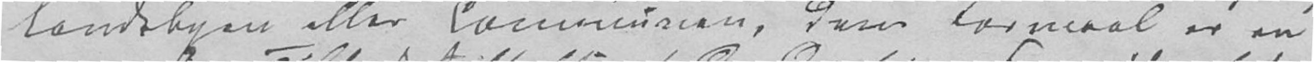Landsbyen eller Communen, deres Formaal er en
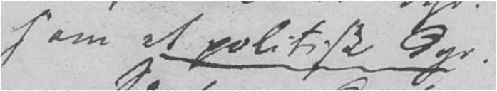som et politisk dyr.
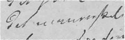det menneskeli-
